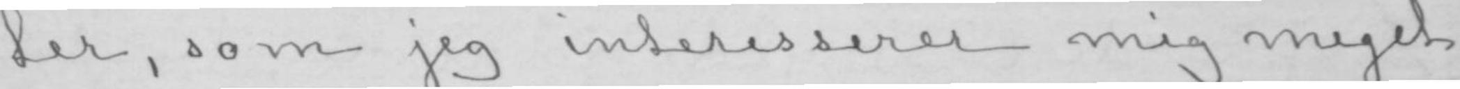ter, som jeg interesserer mig meget
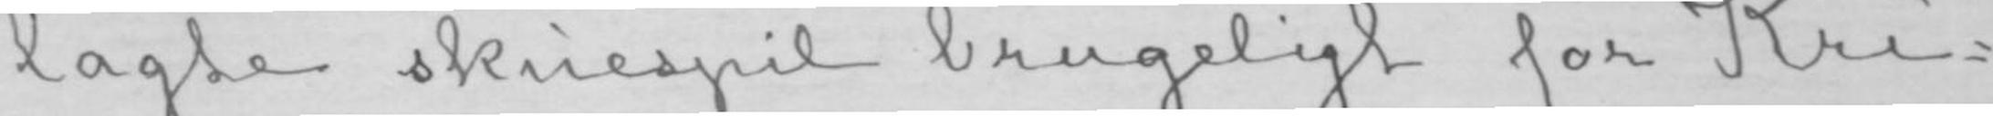lagte skuespil brugeligt for Kri-
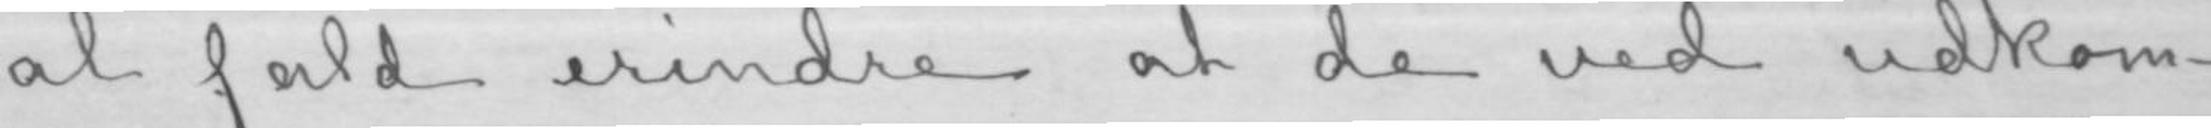al fald erindre at de ved udkom-
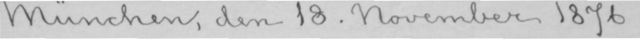München, den 18. November 1876.
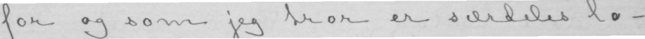for og som jeg tror er særdeles lo-
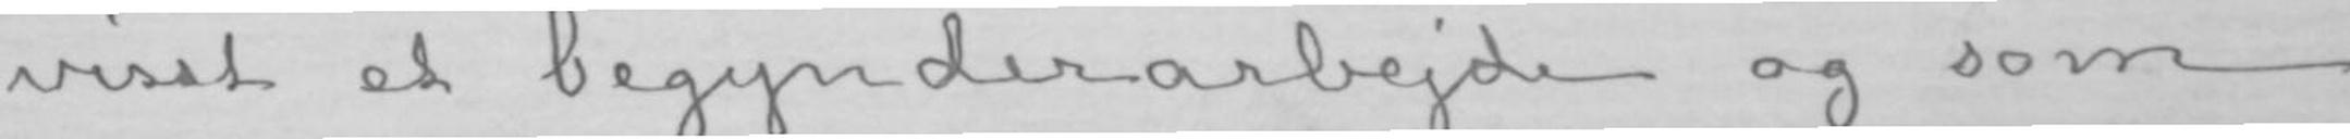visst et begynderarbejde og som
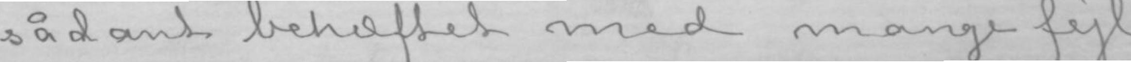sådant behæftet med mange fejl;
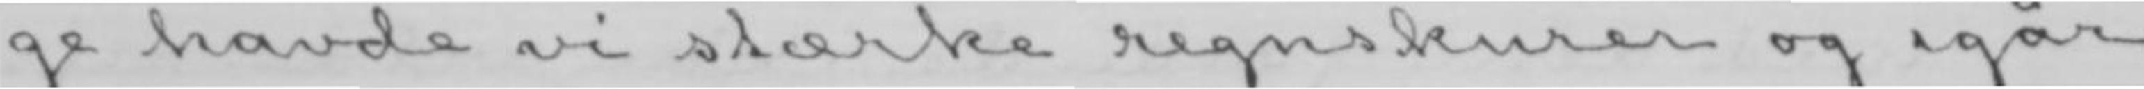ge havde vi stærke regnskurer og igår,
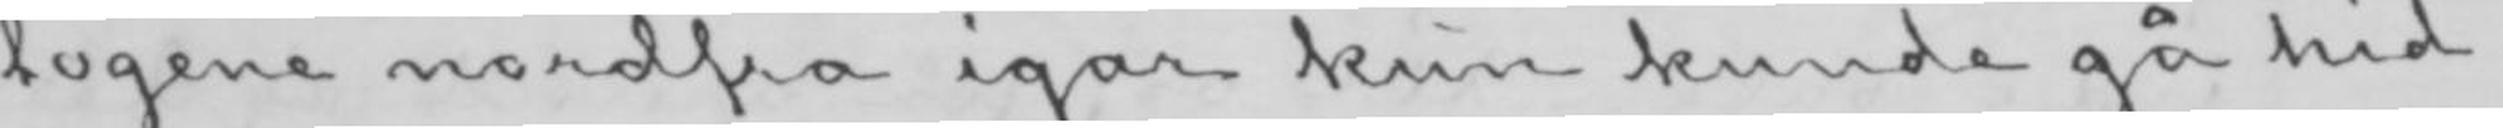togene nordfra igår kun kunde gå hid.
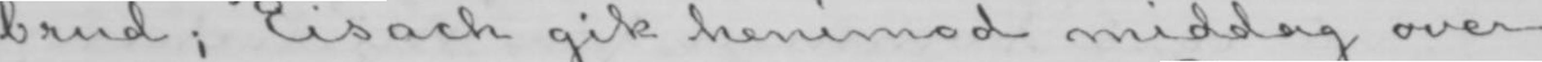brud; Eisach gik henimod middag over
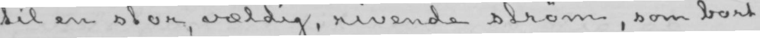til en stor, vældig, rivende strøm, som bort-
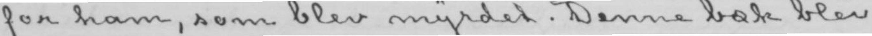for ham, som blev myrdet. Denne bæk blev
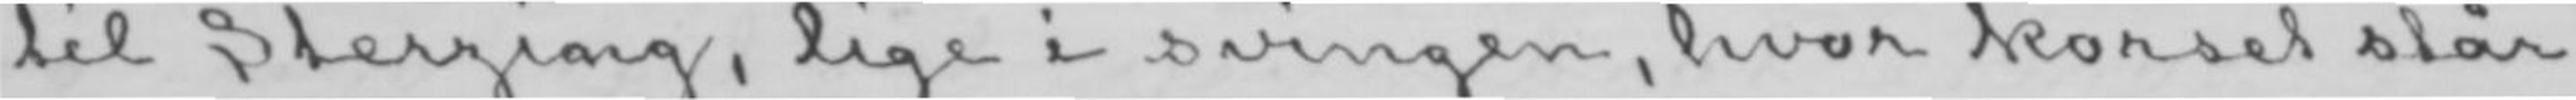til Sterzing, lige i svingen, hvor korset står
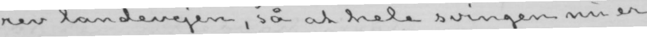rev landevejen, så at hele svingen nu er
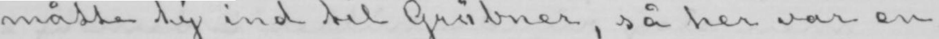måtte ty ind til Gröbner, så her var en
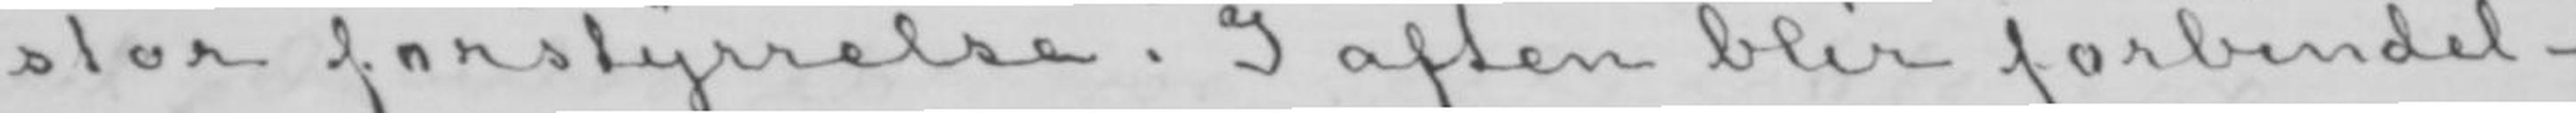stor forstyrrelse. I aften blir forbindel-
